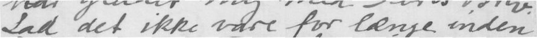Lad det ikke vare for længe inden
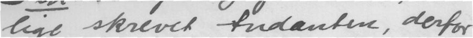lige skrevet Andanten, derfor
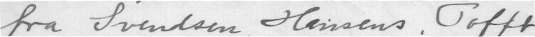fra Svendsen, Hansens, Tofft
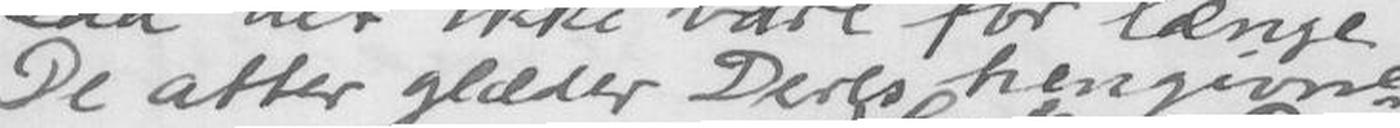De atter glæder Deres hengivne
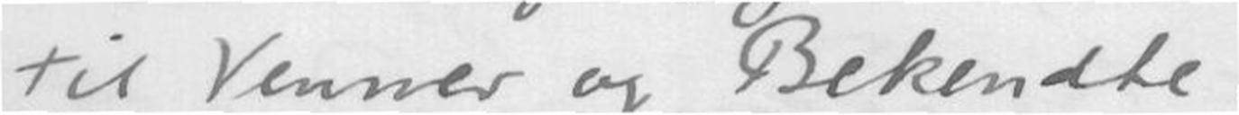til Venner og Bekendte.
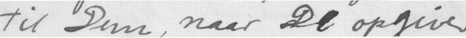til Dem, naar De opgiver
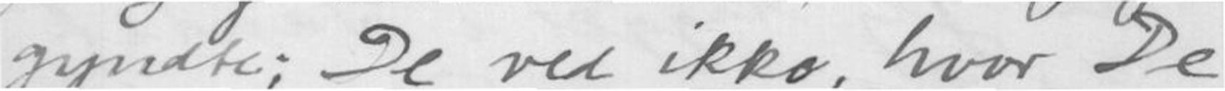gyndte: De ved ikke, hvor De
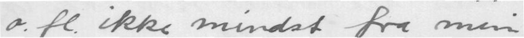o. fl. ikke mindst fra min
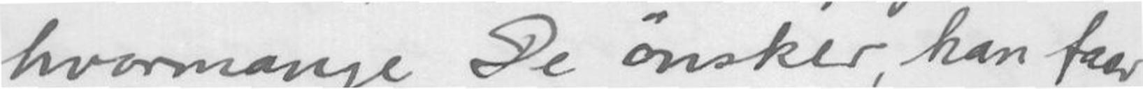hvormange De ønsker, han faar
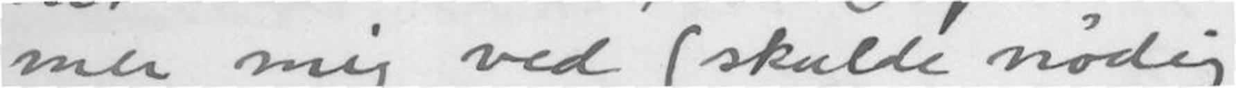mer mig ved (skulde nødig
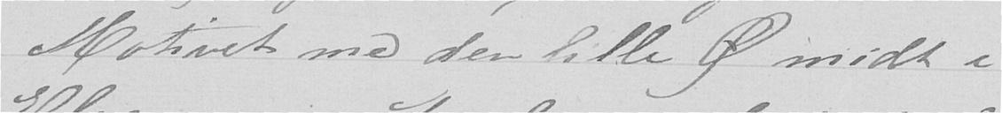Motivet med den lille Ø midt i
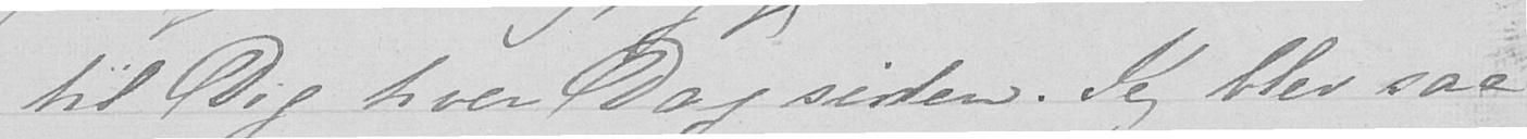til Dig hver Dag siden. Jeg blev saa
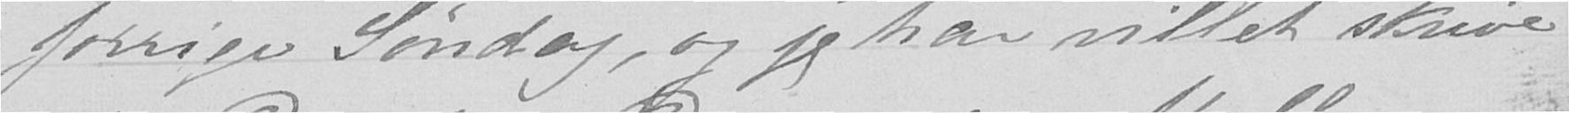forrige Søndag, og jeg har villet skrive
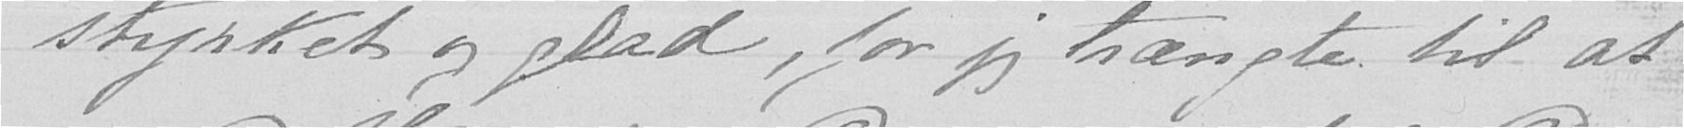styrket og glad, for jeg trængte til at
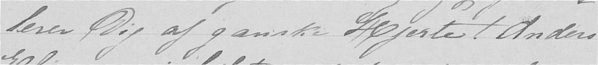lerer Dig af ganske Hjertet! Anders
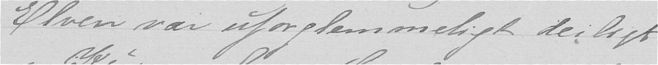Elven var uforglemmeligt deiligt
